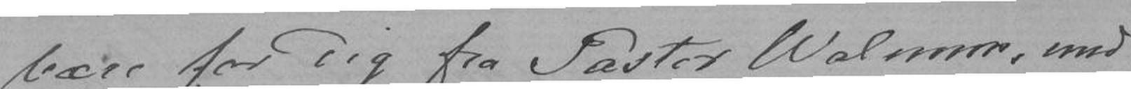bære for Dig fra Pastor Walmans, med
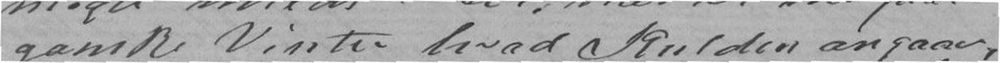ganske Vintre hvad Kulden angaar,
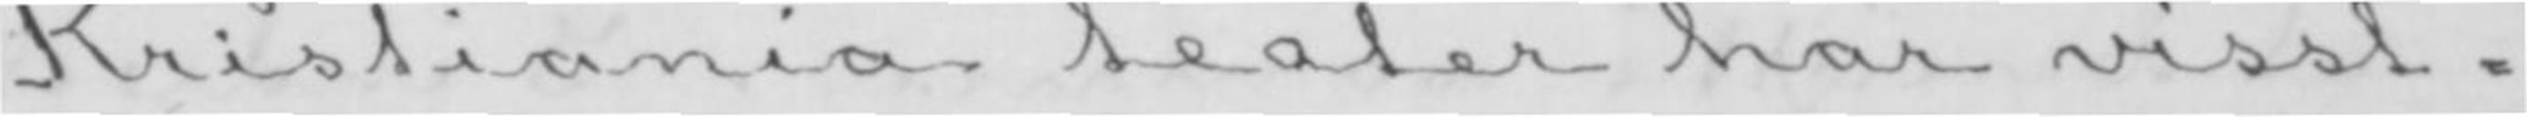Kristiania teater har visst-
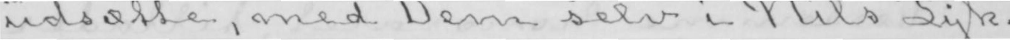udsætte, med Dem selv i Nils Lyk-
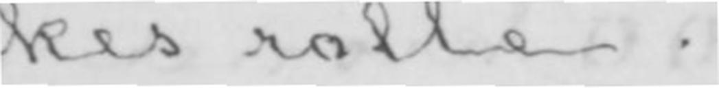kes rolle.
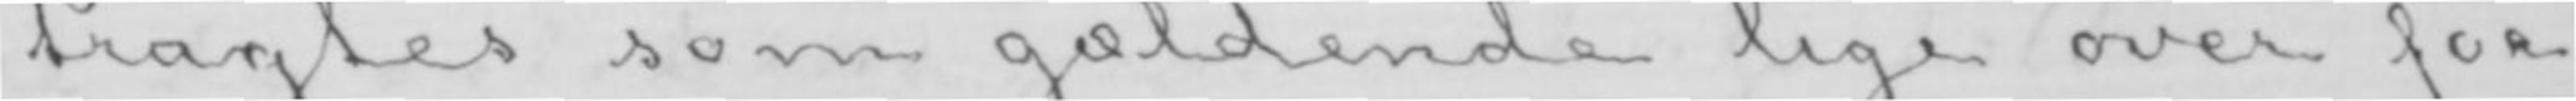tragtes som gældende lige over for
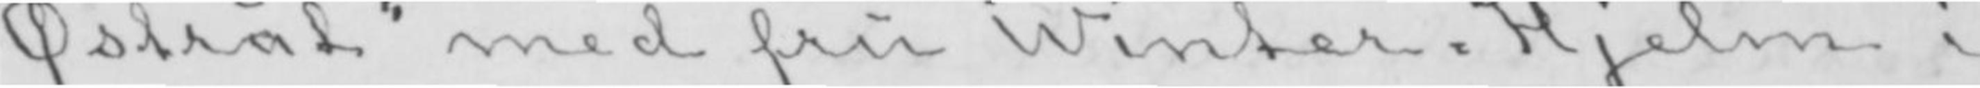Østråt» med fru Winter-Hjelm i
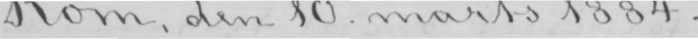Rom, den 10. marts 1884.
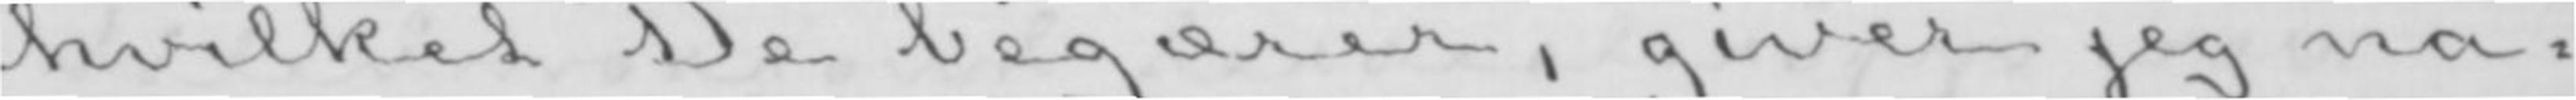hvilket De begærer, giver jeg na-
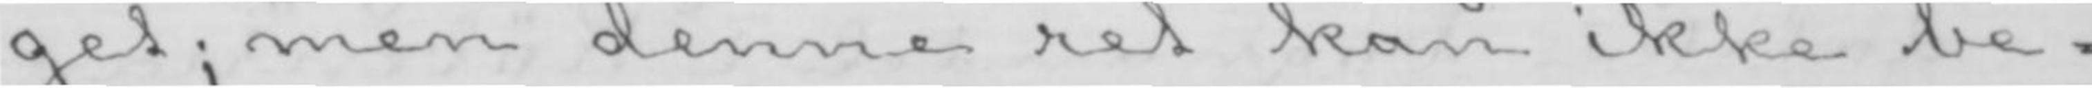get; men denne ret kan ikke be-
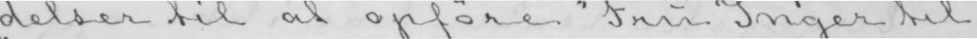delser til at opføre «Fru Inger til
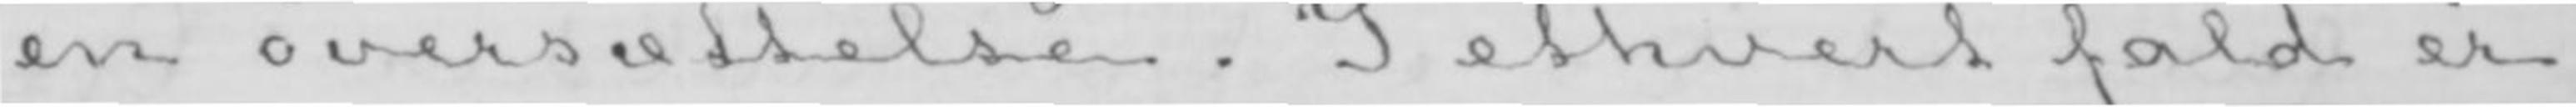en oversættelse. I ethvert fald er
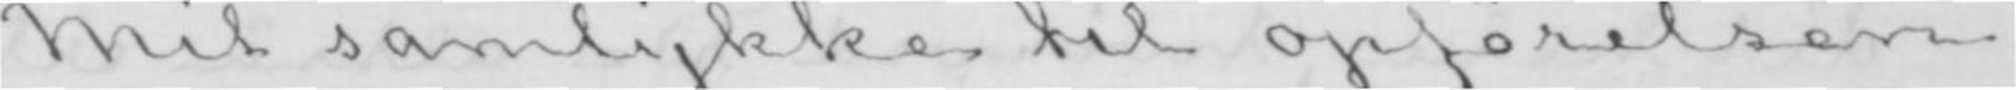Mit samtykke til opførelsen,
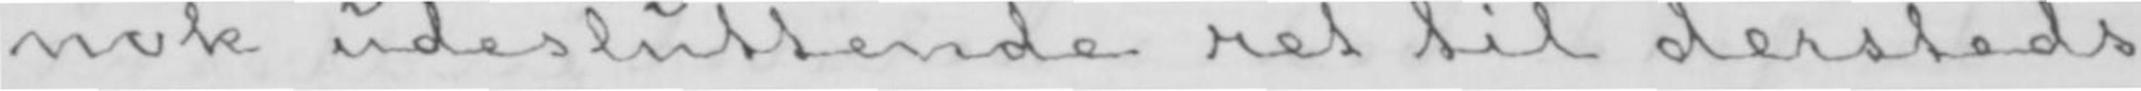nok udesluttende ret til dersteds
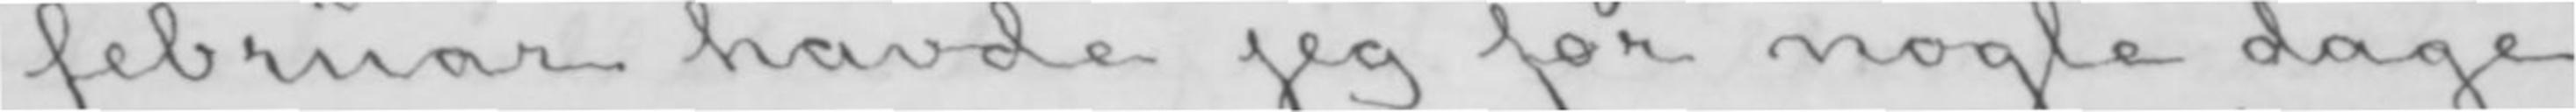februar havde jeg for nogle dage
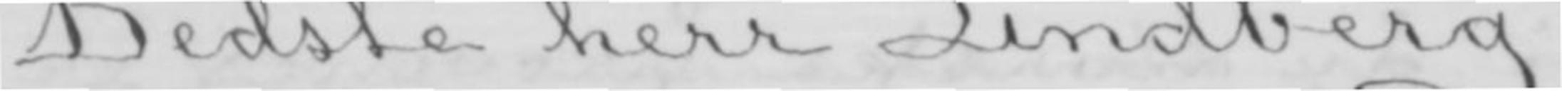Bedste herr Lindberg!
